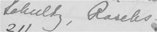Schultz, Raschs.
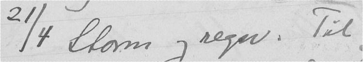21/4 Storm og regn. Til
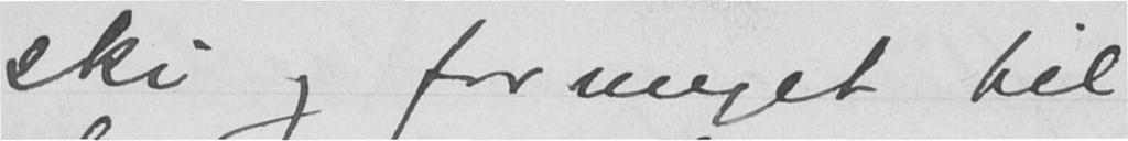ski og for meget til
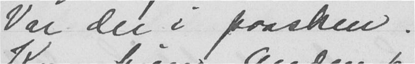Var der i paasken.
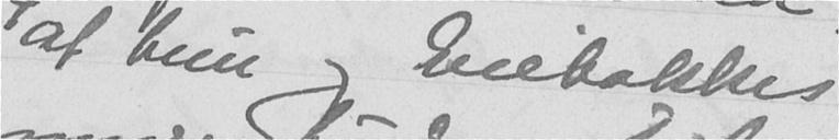at hun og Eiebakkes
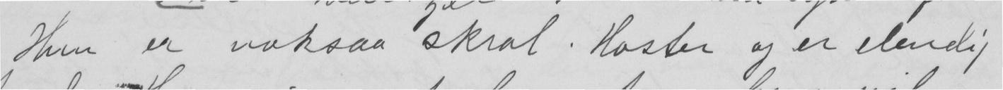Hun er noksaa skral. Hoster og er elendig
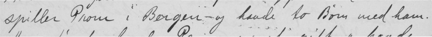spiller Prom i Bergen - og havde to Børn med ham.
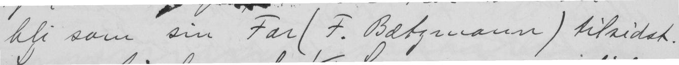bli som sin Far (F. Bætzmann) tilsidst.
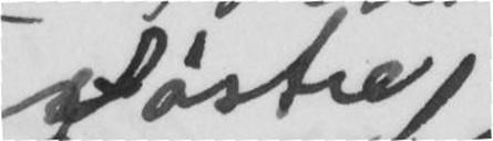Søstre,
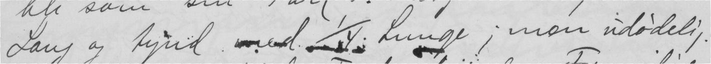Lang og tynd med 1/4 Lunge; men udødelig.
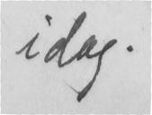idag.
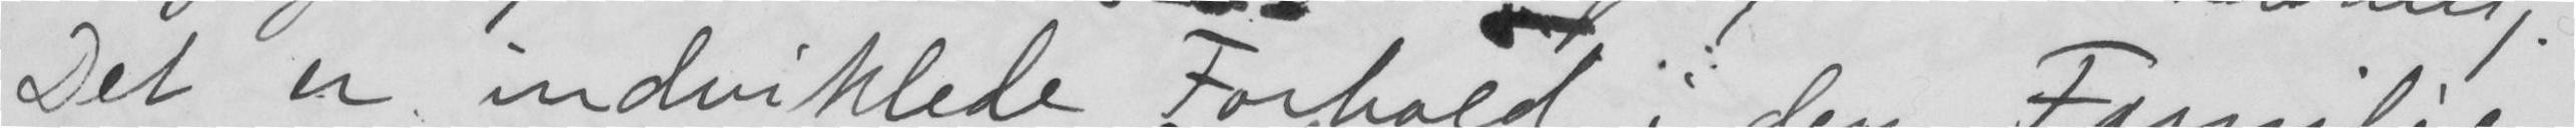Det er indviklede Forhold i den Famlie.
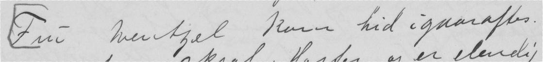Fru Wentzel kom hid igaaraftes.
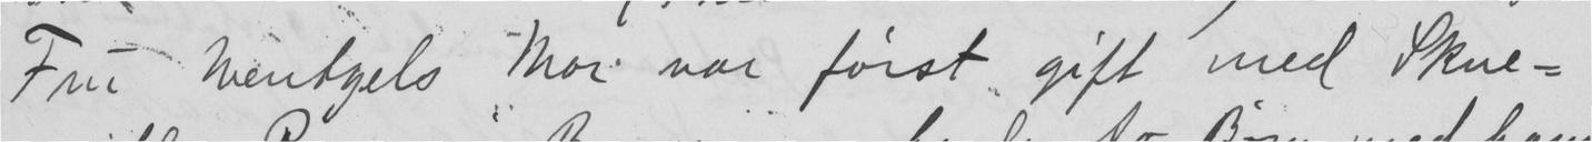Fru Wentzels Mor var først gift med Skue-
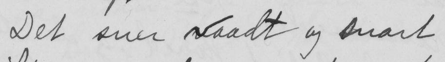Det sner vaadt og snart
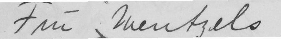Fru Wentzels
